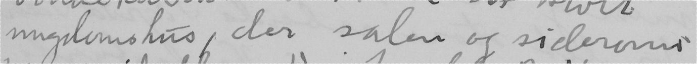ungdomshus, der salen og siderom
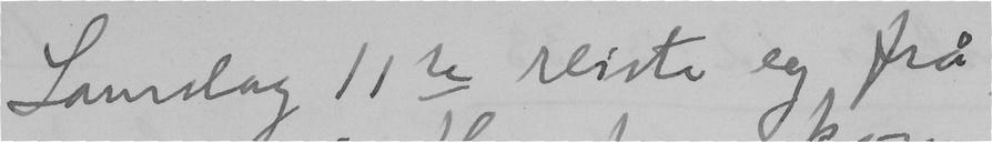Laurdag 11te reiste eg frå
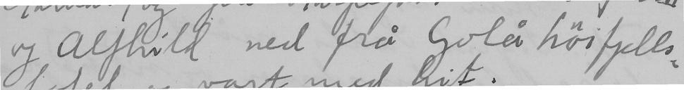og Alfhild ned fra Golå höifjells-
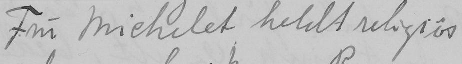Fru Michelet heldt religiös
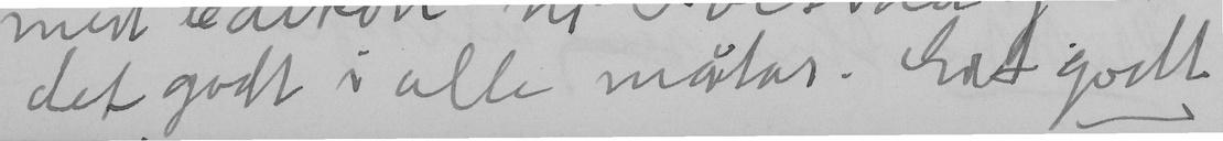det godt i alle måtar. Et godt
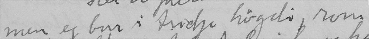men eg bur i tridje högdi, rom
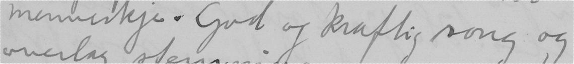menneskje. God og kraftig song og
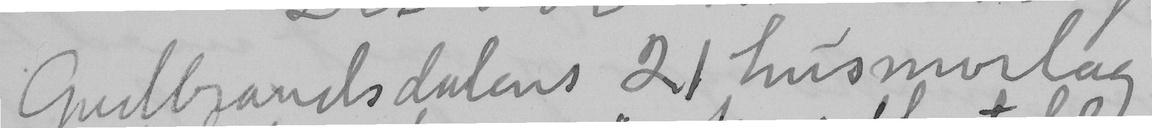Guldbrandsdalens 21 husmorlag
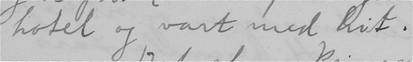hotel og vart med hit.
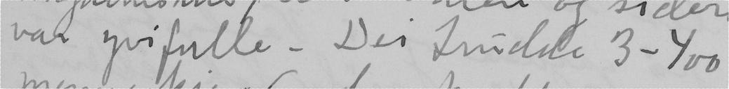var yvifulle. Dei trudde 3-400
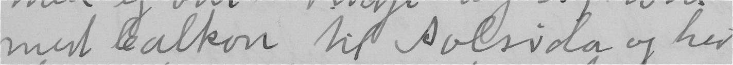med balkon til solsida og hev
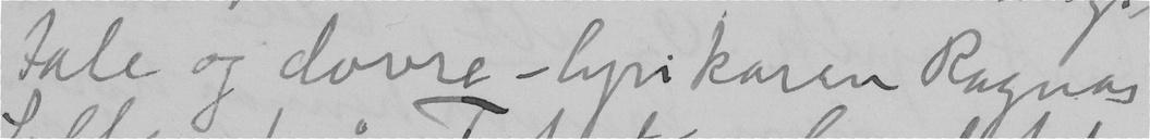tale og dovre-lyrikaren Ragnar
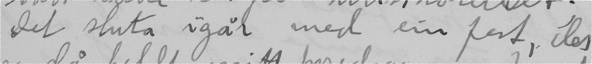Det sluta igår med ein fest, der
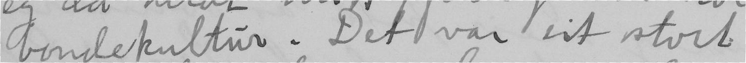bondekultur. Det var eit stort
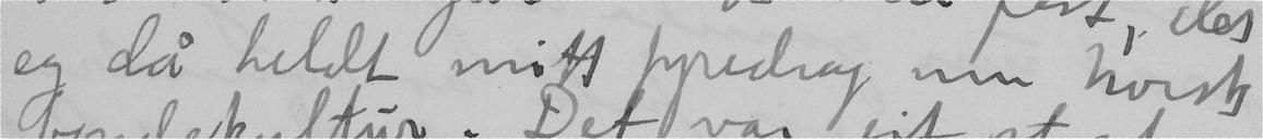eg då heldt mitt fyredrag um norsk
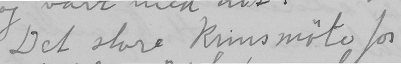Det store Krinsmöte for
